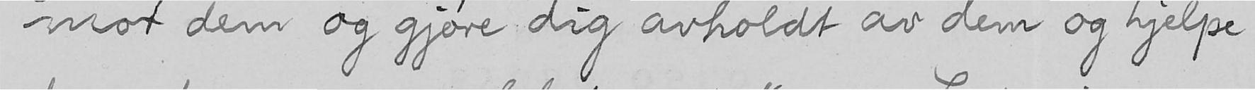mot dem og gjøre dig avholdt av dem og hjelpe
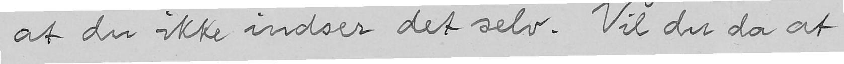at du ikke indser det selv. Vil du da at
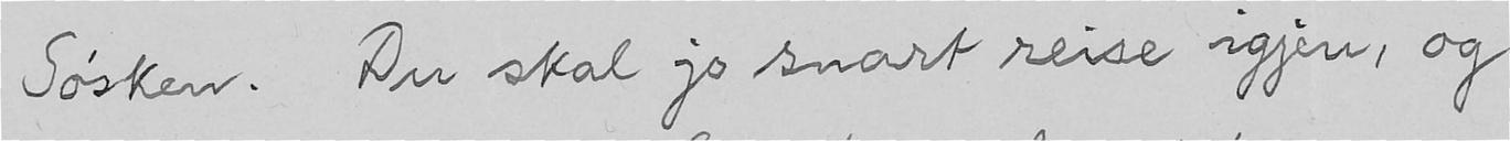Søsken. Du skal jo snart reise igjen, og
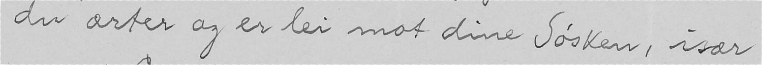du ærter og er lei mot dine Søsken, især
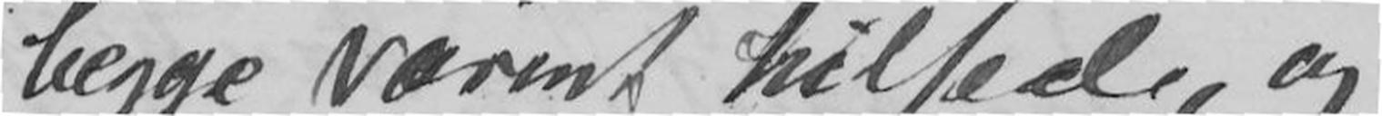begge varmt hilsede, og
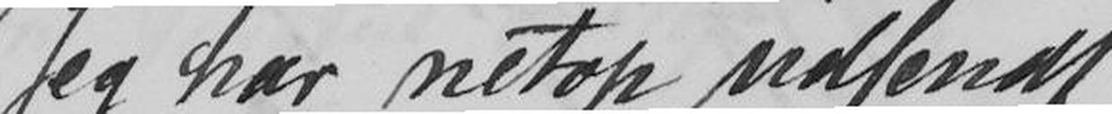Jeg har netop udsendt
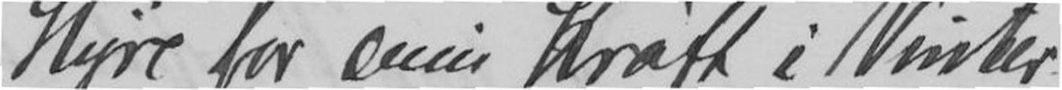Hyre for sin Kraft i Vinter.
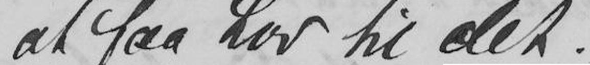af faa Lov til det.
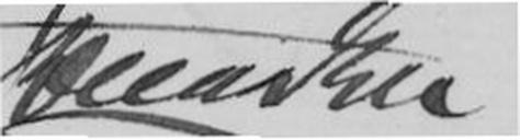Jonas Lie.
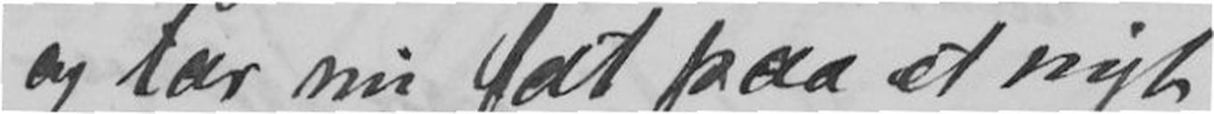og tar nu fat paa et nyt
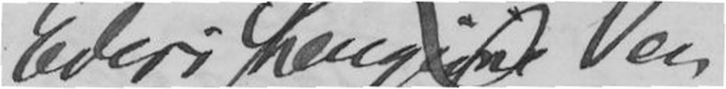Eders hengivne Ven
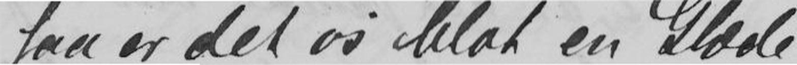saa er det os blot en Glæde
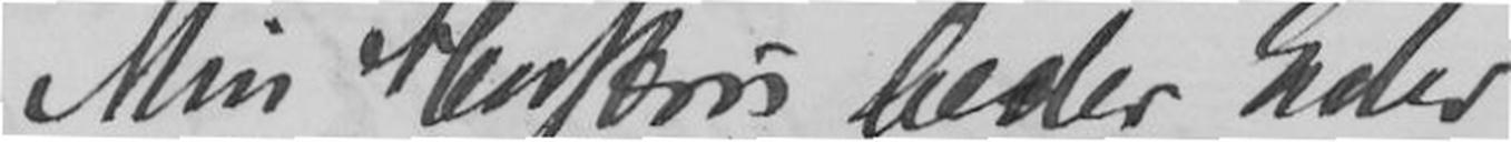Min Hustru beder Eder
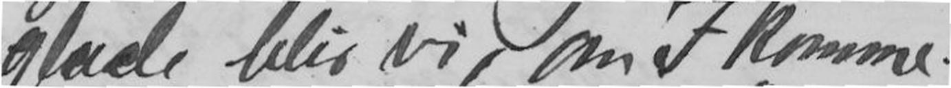glade blir vi, om I komme
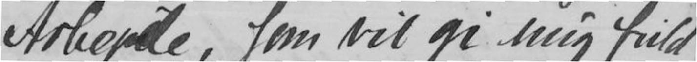Arbejde, som vil gi mig fuld
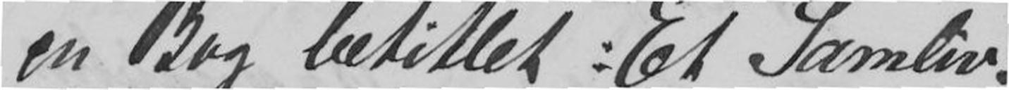en Bog bestillet: Et Samliv.
